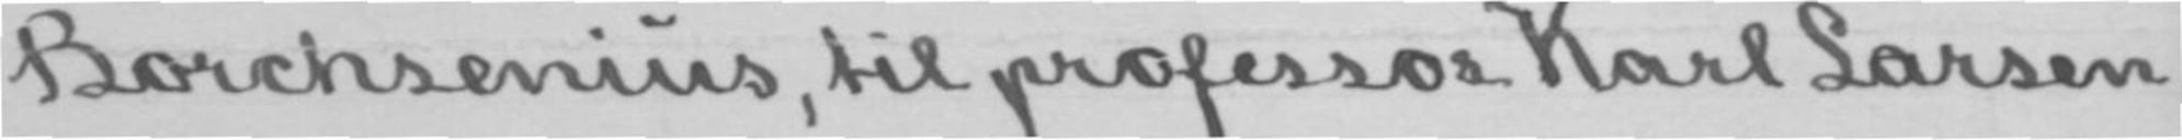Borchsenius, til professor Karl Larsen,
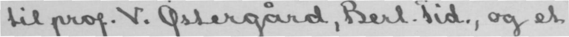til prof. V. Østergård, Berl. Tid., og et
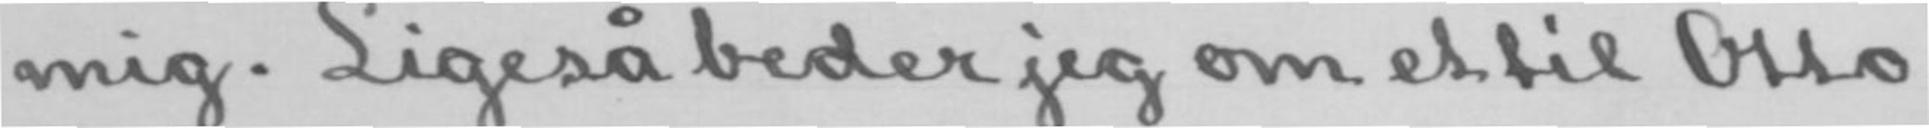mig. Ligeså beder jeg om et til Otto
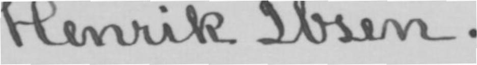Henrik Ibsen.
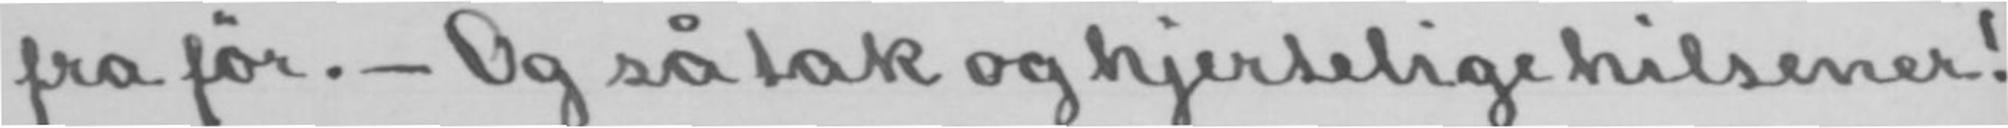fra før.- Og så tak og hjertelige hilsener!
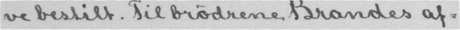ve bestilt. Til brødrene Brandes af-
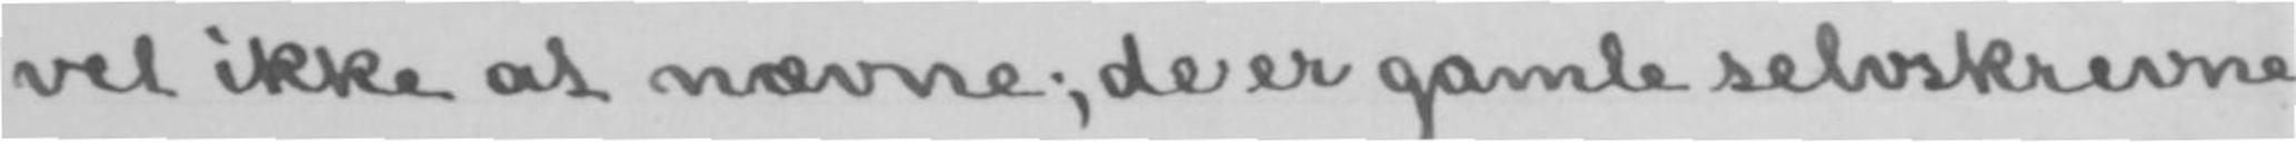vel ikke at nævne; de er gamle selvskrevne
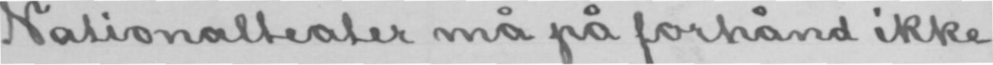Nationalteater må på forhånd ikke
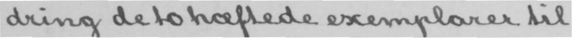dring de to hæftede exemplarer til
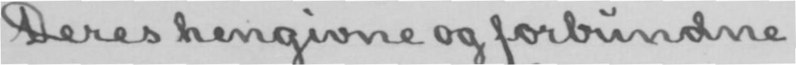Deres hengivne og forbundne
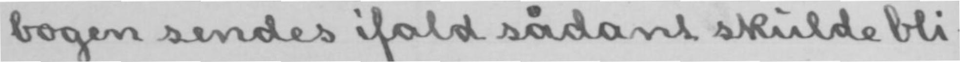bogen sendes ifald sådant skulde bli-
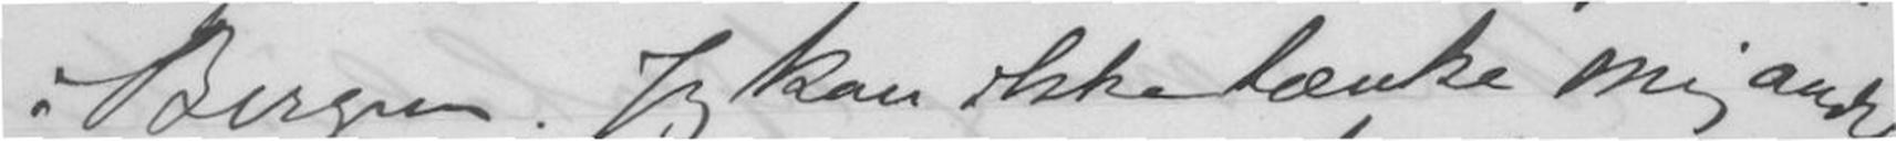i Bergen. Jeg kan ikke tænke mig andet
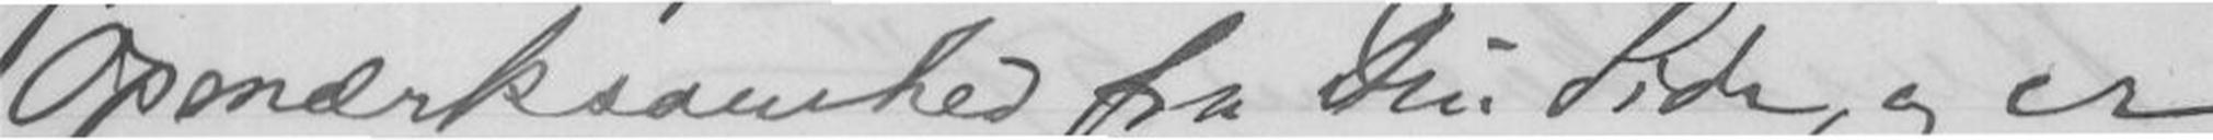Opmærksomhed fra Din Side, og er
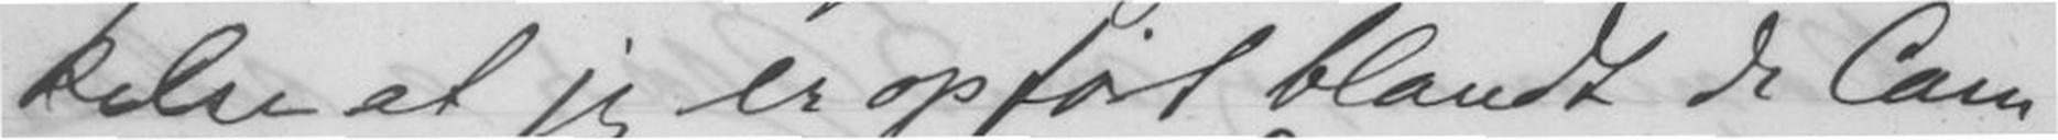kelse at jeg er opført blandt de Com-
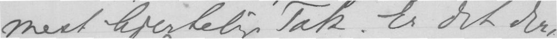mest hjertelige Tak. Er det der-
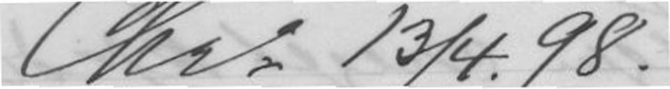Chr. 13/4.98.
